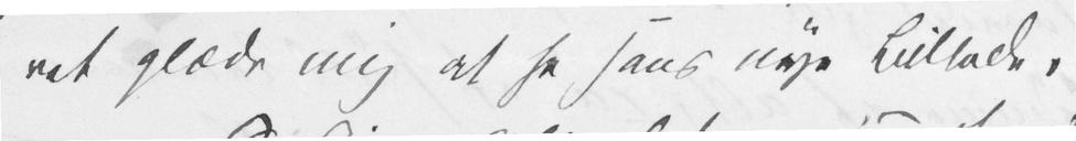ret glæde mig at se hans nye Billede,
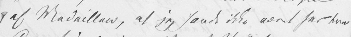af Medaillen, at jeg havde ikke været her tre
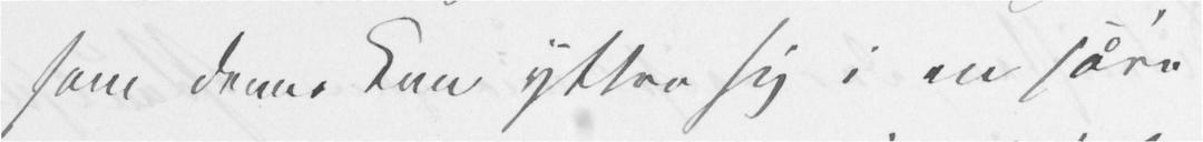som denne kan yttre sig i en såre
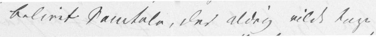belivet Samtale, der aldrig vilde tage
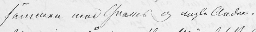sammen med Grams og nogle Andre.
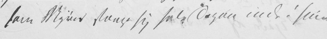som Myrer stænge sig hele Dagen inde i sine
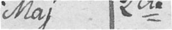Maj 2de
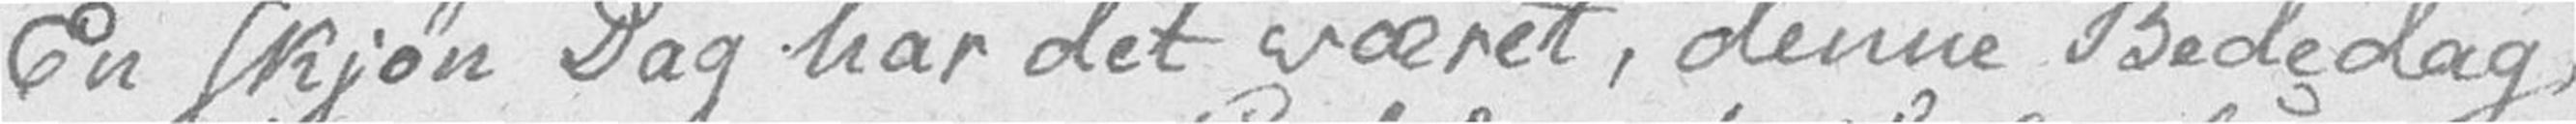En skjøn Dag har det været, denne Bededag,
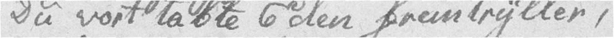Du vort tabte Eden fremtryller,
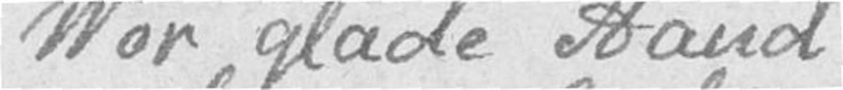Vor glade Aand
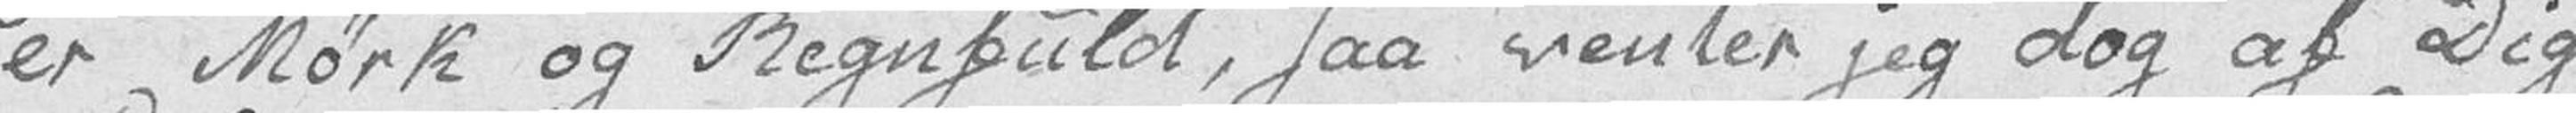er Mørk og Regnfuld, saa venter jeg dog af Dig
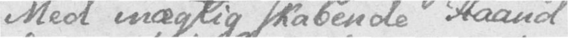Med mægtig skabende Haand
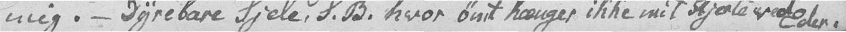mig. – Dyrebare Sjele, S. B. hvor ømt hænger ikke mit Hjerte ved Eder.
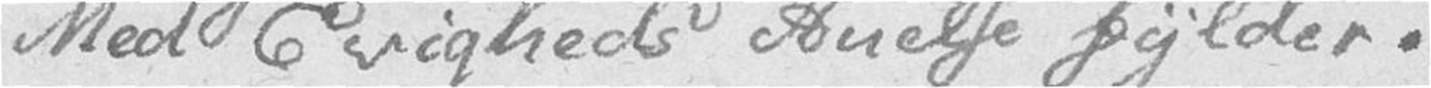Med Evigheds Anelse fylder.
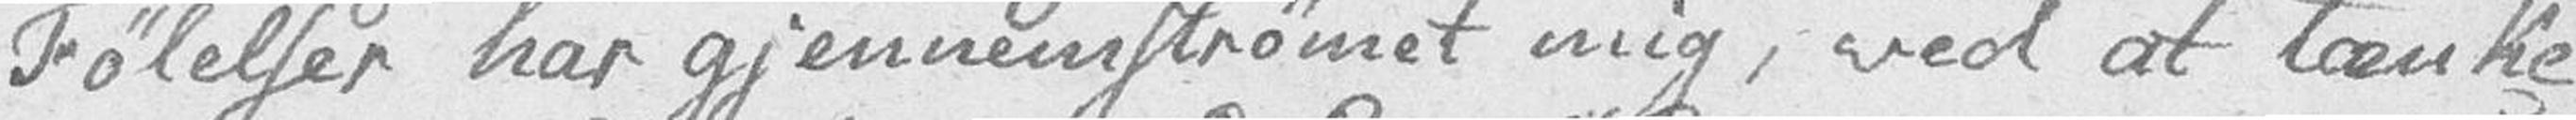Følelser har gjennemstrømet mig, ved at tænke
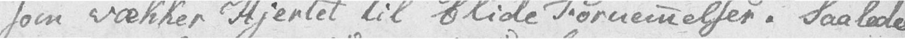som vækker Hjertet til blide Fornemmelser. Saaledes
